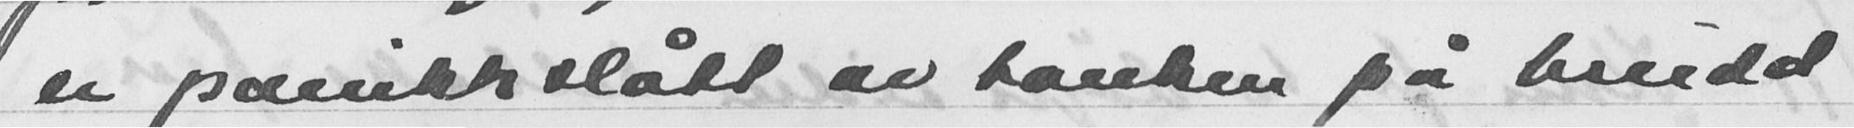er panikkslått av tanken på brudd
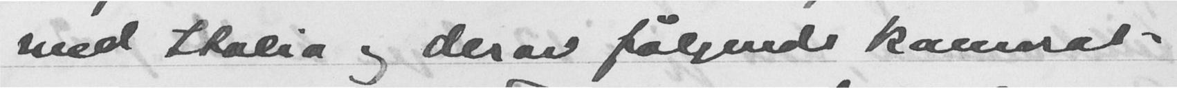med Italia og derav følgend kamerat-
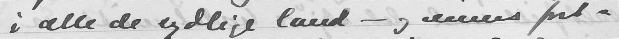i alle de sydlige land - og renees fort-
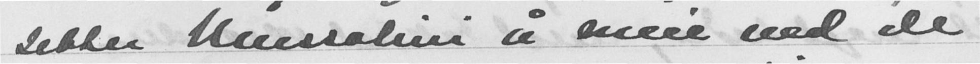Setter Mussolini å meie ned de
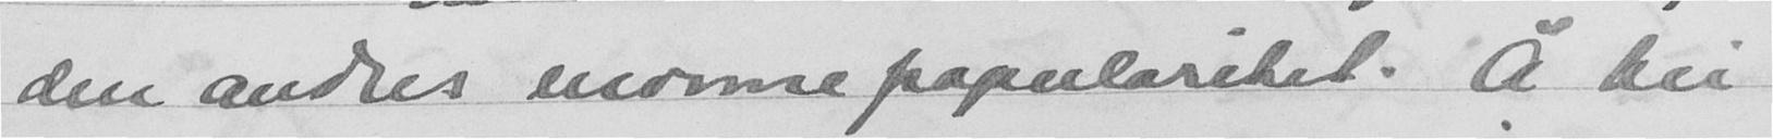den andres enorme papularitet. Å bli
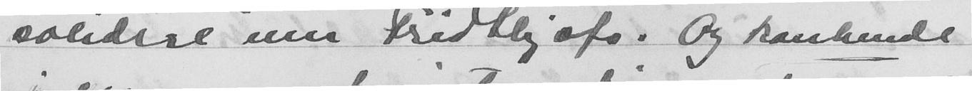solidere enn Fridthjofs. Og kanhende
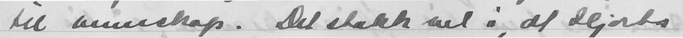til vennskap. Det stakk vel i, at Hjorts
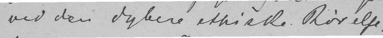ved den dybere ethiske Rørelse,
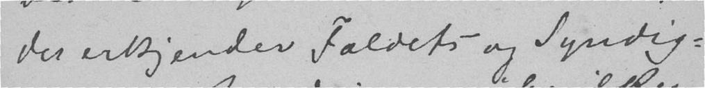der erkjender Faldets og Syndig-
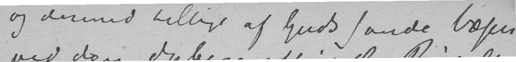og dermed tillige af Guds sande Væsen
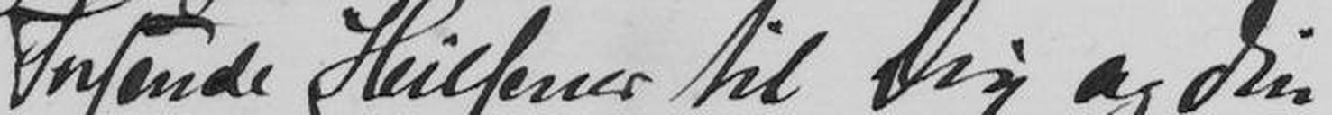Tusende Hilsener til Dig og din
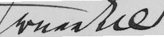Jonas Lie.
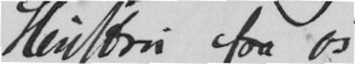Hustru fra os
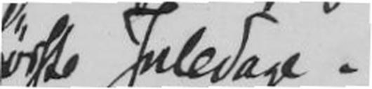første Juledage.
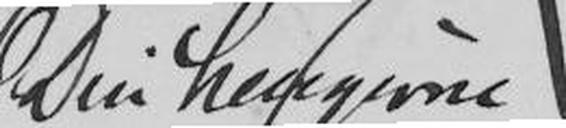Din hengivne
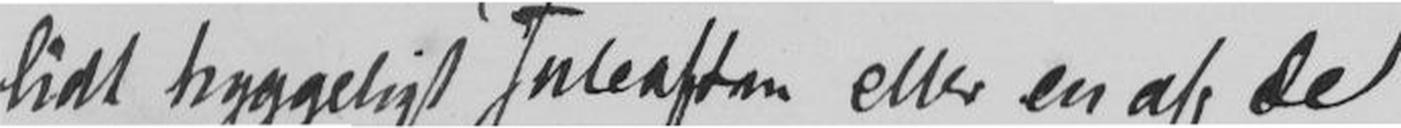lidt hyggeligt Juleaften eller en af de
